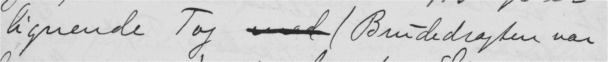lignende Tog  (Brudedragten var
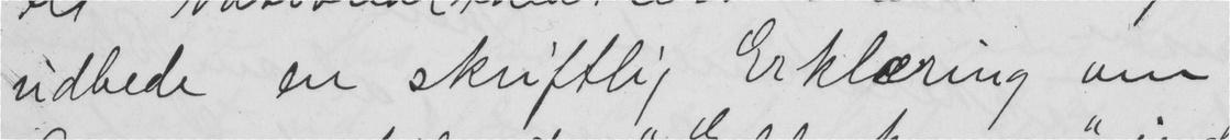udbede en skriftlig Erklæring om
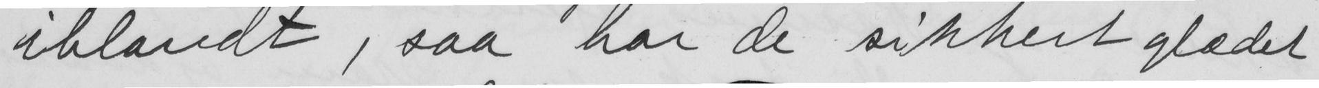iblandt, saa har de sikkert glædet
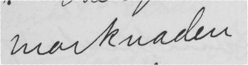marknaden
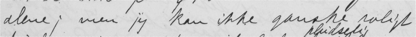alene; men jeg kan ikke ganske roligt
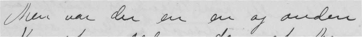Men var der en er og anden
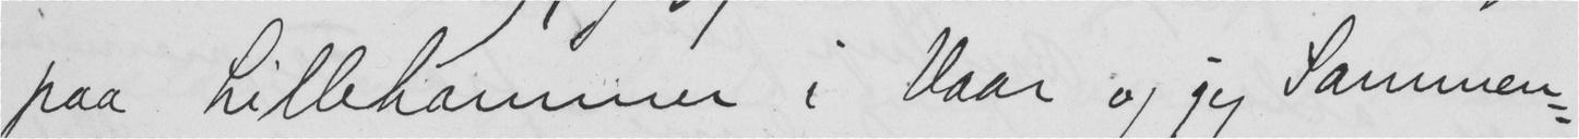paa Lillehammer i Vaar og jeg Sammen-
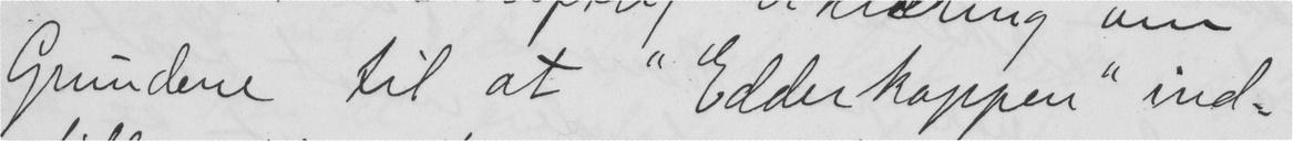Grundene til at "Edderkoppen" ind-
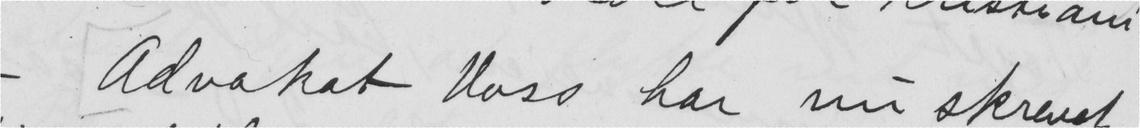— Advakat Voss har nu skrevet
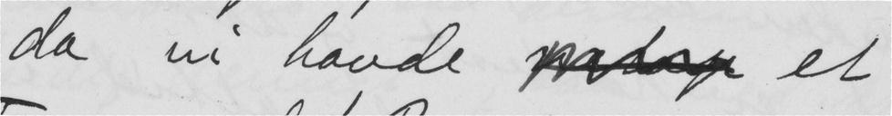da vi havde  et
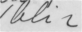blir
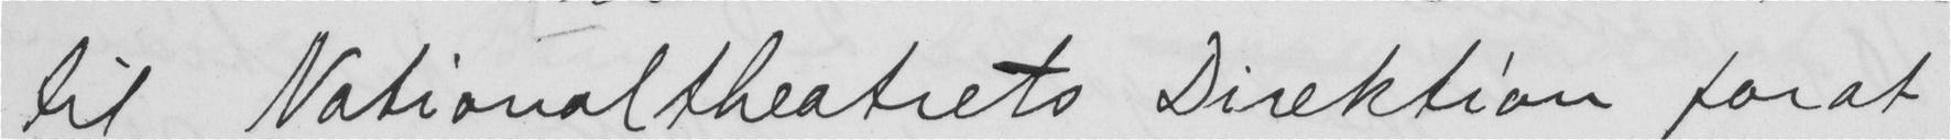til Nationaltheatrets Direktion forat
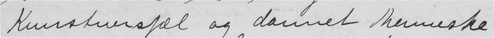Kunstnersjæl og dannet Menneske
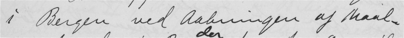i Bergen ved Aabningen af Maal-
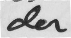der
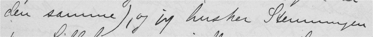den samme), og jeg husker Stemningen
